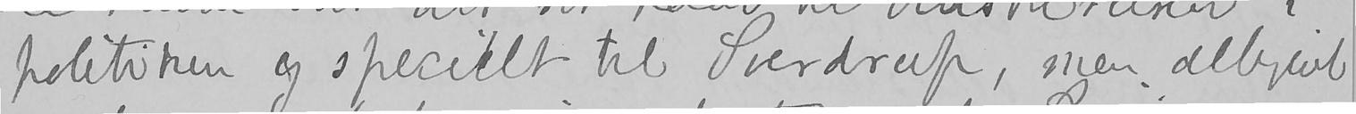politiken og specielt til Sverdrup, men alligevel
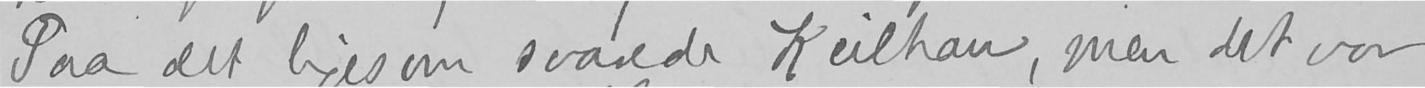Paa det ligesom svarede Keilhau, men det var
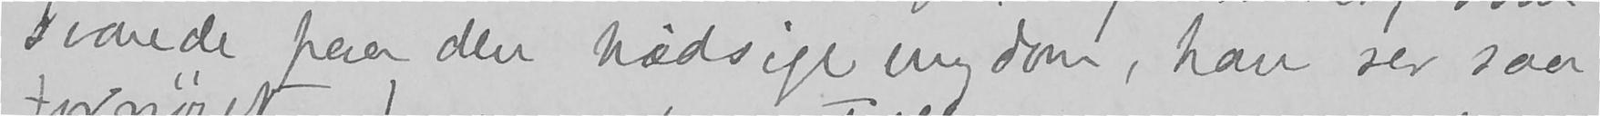svarede paa den hidsige ungdom, han ser saa
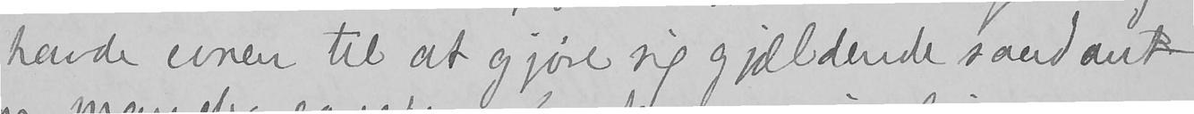havde evnen til at gjøre sig gjældende saadant
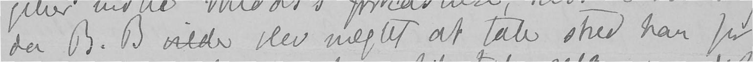da B. B. vilde blev nægtet at tale stred han for
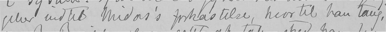gelser indtil Midas's forkastelse, hvortil han taug,
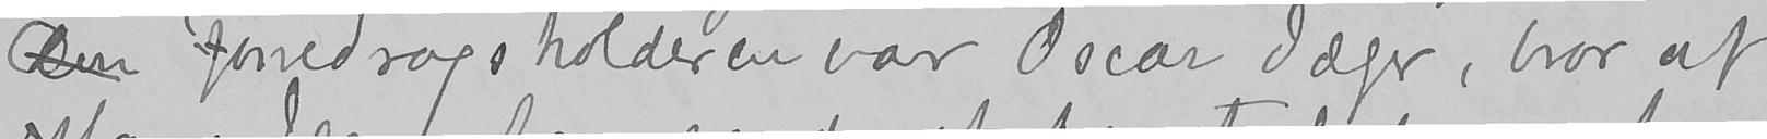Den Foredragsholderen var Oscar Jæger, bror af
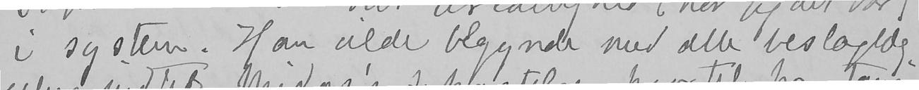i system. Han vilde begynde med alle beslaglæg-
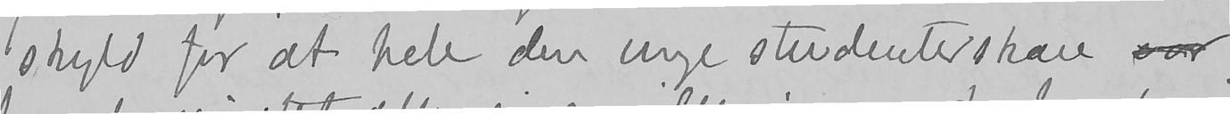skyld for at hele den unge studenterskare var
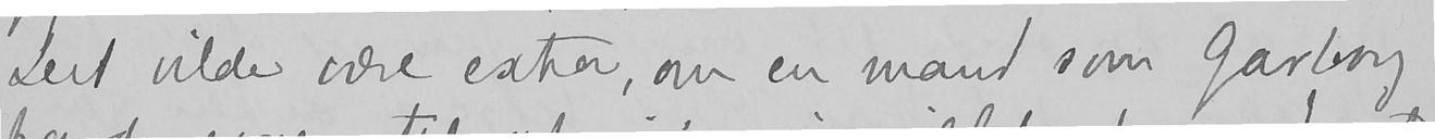Det vilde være extra, om en mand som Garborg
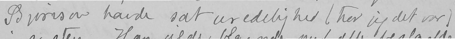Bjørnson havde sat uredelighed (tror jeg det var)
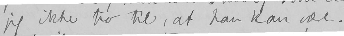jeg ikke tro til, at han kan være.
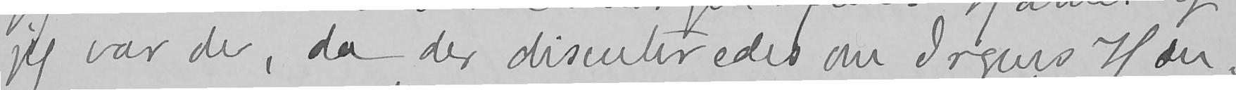jeg var der, da der discuteredes om Irgens Han-
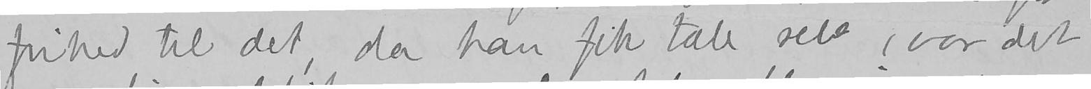frihed til det, da han fik tale selv, var det
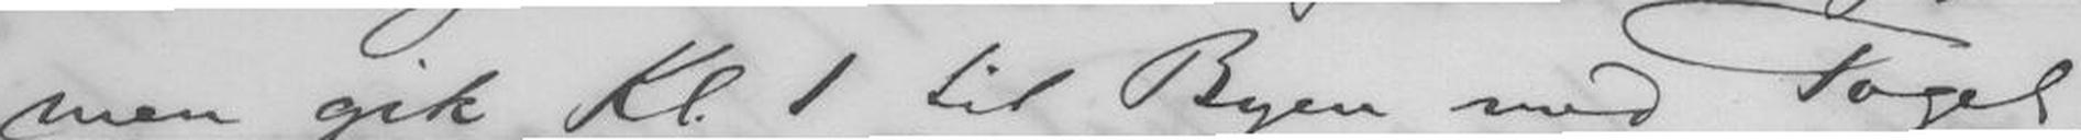men gik Kl.1 til Byen med Toget,
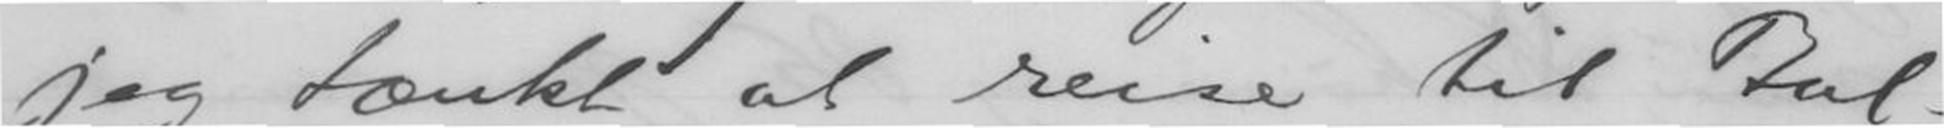jeg tænkt at reise til Bul-
